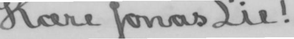Kære Jonas Lie!
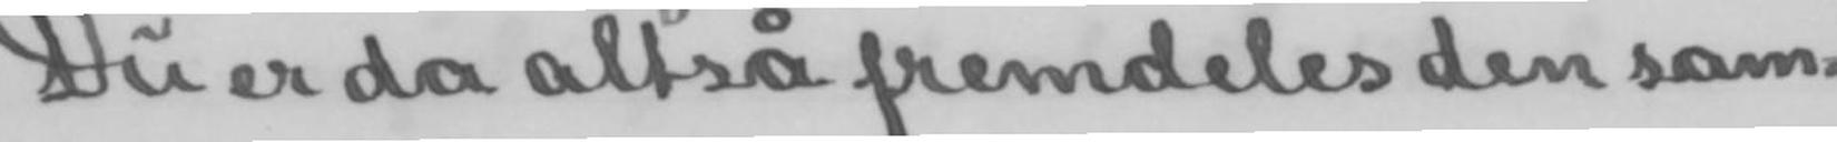Du er da altså fremdeles den sam-
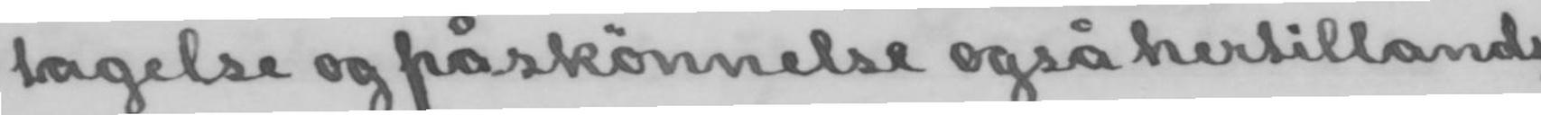tagelse og påskønnelse også hertillands,
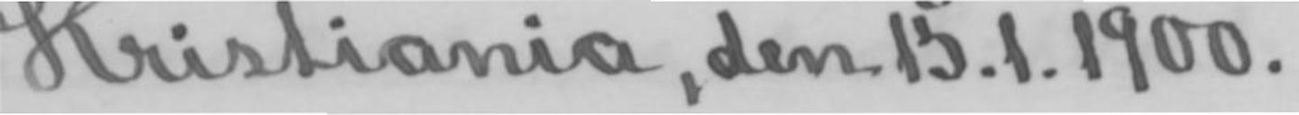Kristiania, den 15.1.1900.
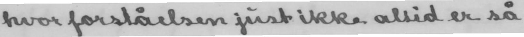hvor forståelsen just ikke altid er så
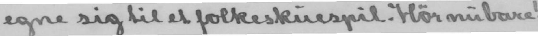egne sig til et folkeskuespil. Hør nu bare!
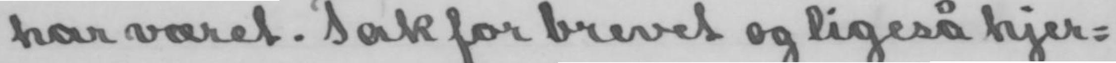har været. Tak for brevet og ligeså hjer-
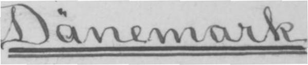Dänemark.
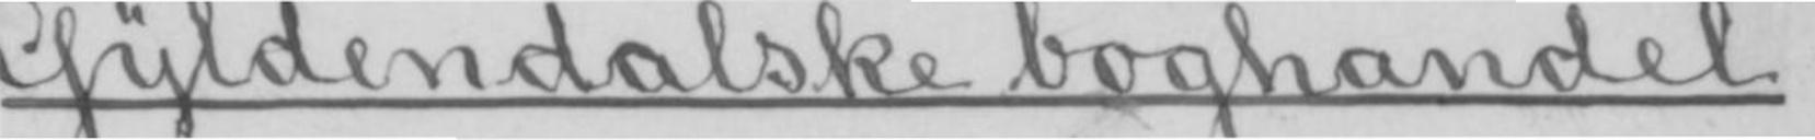Gyldendalske boghandel.
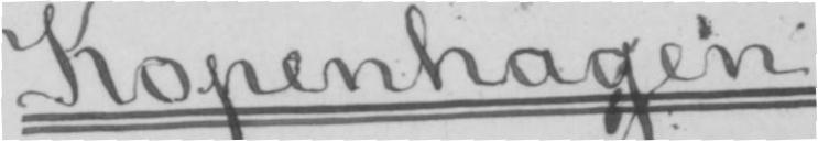Kopenhagen.
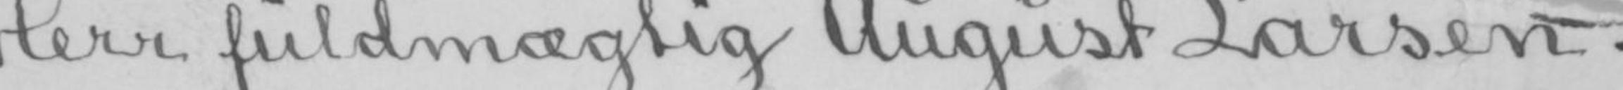Herr fuldmægtig August Larsen.
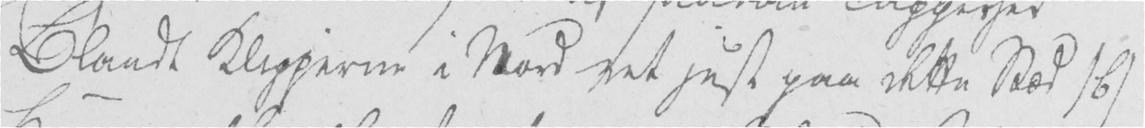Blandt Klipperne i Nord ret just paa dette Stæd / b /
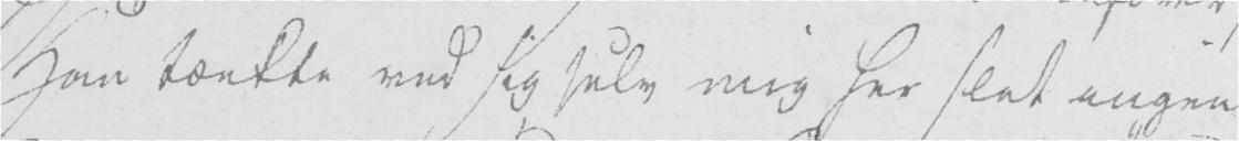Han tænkte ved sig selv mig her slet ingen
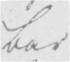bar
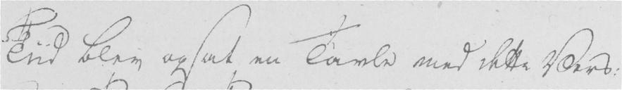Tiid blev opsat en Tavle med dette Værs:
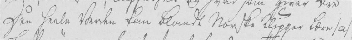Den heele Verden kan blandt Norske Klipper lære / a /
